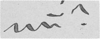nu?
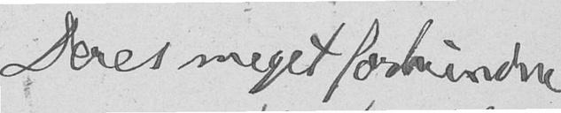Deres meget forbundne
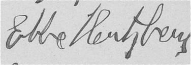Ebbe Hertzberg
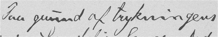Paa grund af trykningens
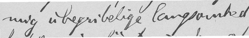mig ubegribelige langsomhed
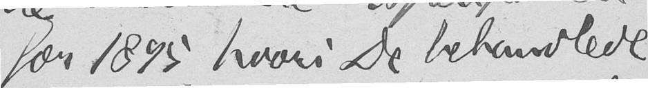for 1895, hvori De behandlede
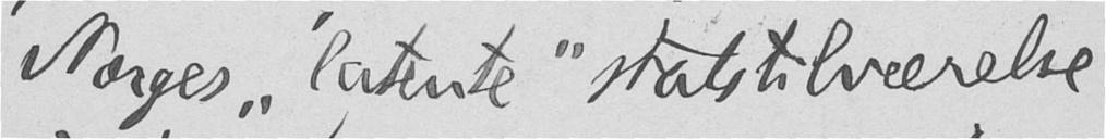Norges "latente" statstilværelse
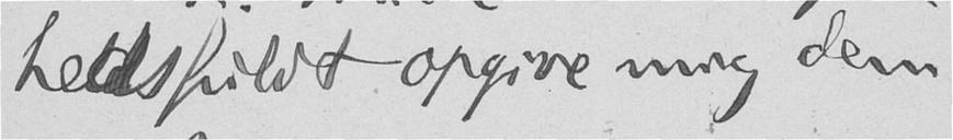hedsfuldt opgive mig dem
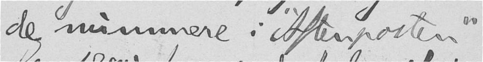de nummere i "Aftenposten"
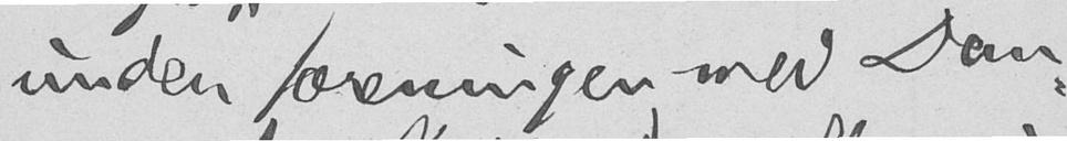under foreningen med Dan-
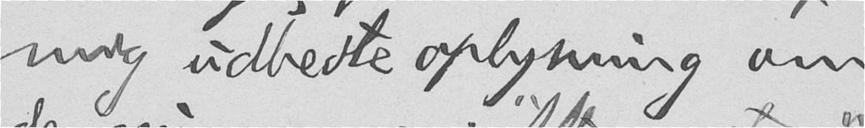mig udbedte oplysning om
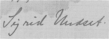Sigrid Undset.
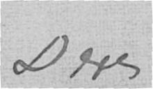Deres
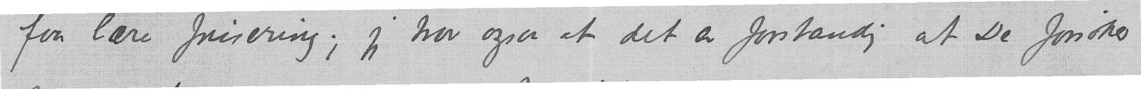faa lære frisering; jeg tror ogsaa at det er forstandig at De forsøker
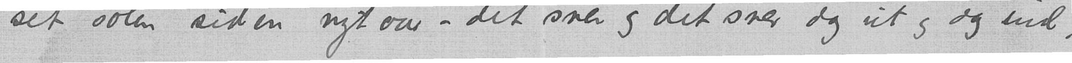set solen siden nytaar – det sner og det sner dag ut og dag ind,
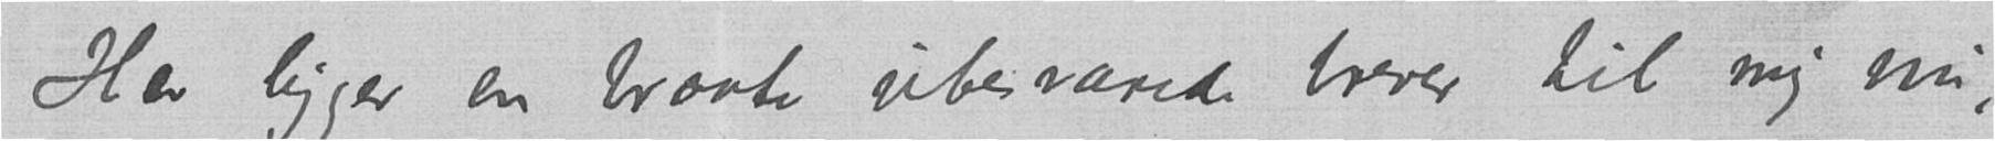Her ligger en braate ubesvarede brever til mig nu,
